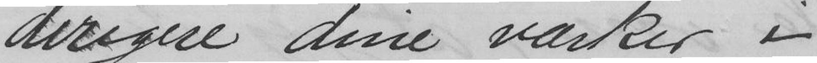dirigere dine værker i
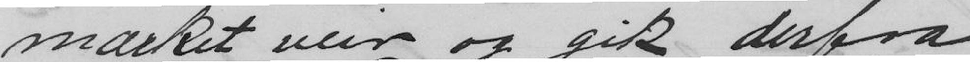mærket veir og gik derfra
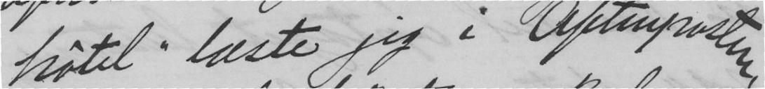hôtel læste jeg i Aftenposten
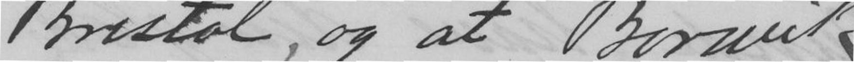Bristol, og at Borwik
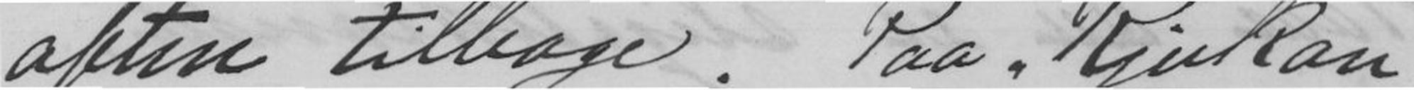aften tilbage. Paa Rjukan
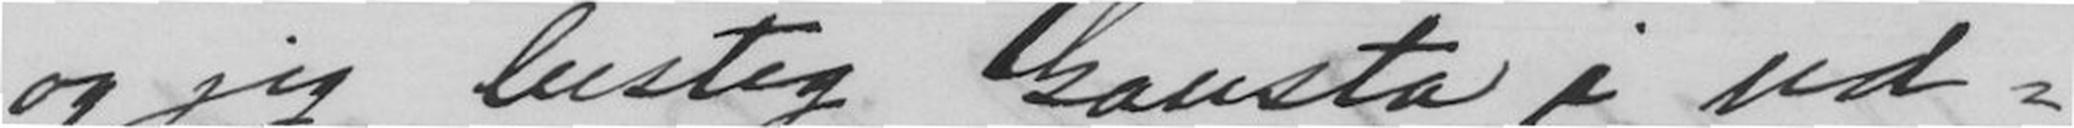og jeg besteg Gausta i ud-
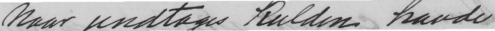Naar undtages kulden havde
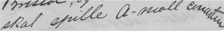skal spille A-moll concerten.
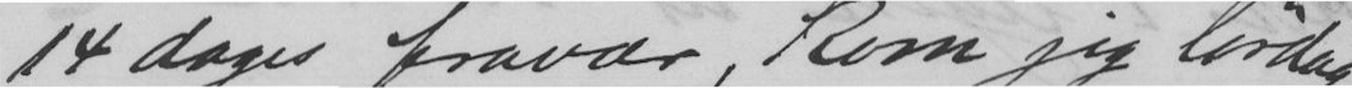14 dages fravær, kom jeg lørdag
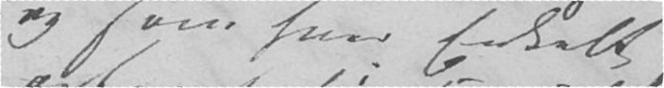og som hver enkelt
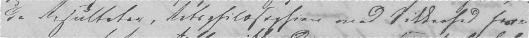de Resultater, Retsphilosophien med Sikkerhed for-
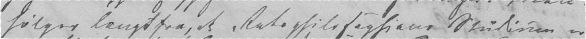følger langtfra, et Retsphilosophiens Studium er
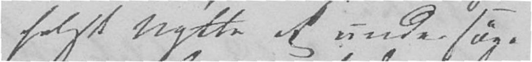helst Nytte at undersøge
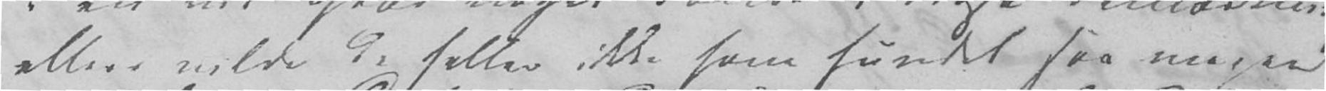ellers vilde de heller ikke have fundet saa megen
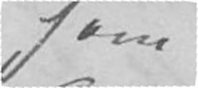som
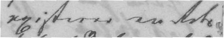existerer en Rets-
